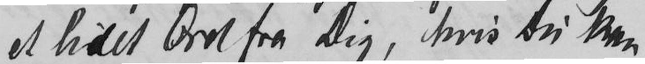et lidet Ord fra Dig, hvis Du kan
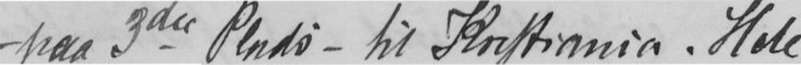-paa 3die Plads - til Kristiania. Hele
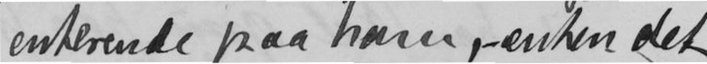enterende paa ham, - enten det
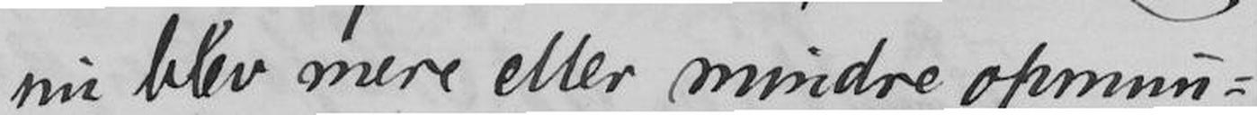nu blev mere eller mindre opmun-
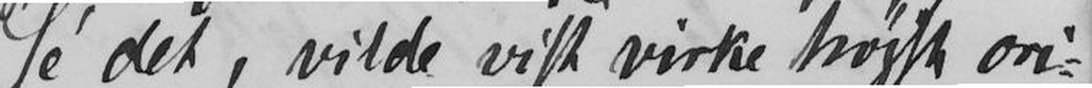se det, vilde vist virke højst ori-
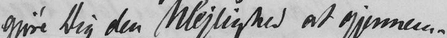gjøre Dig den Ulejlighed at gjennem-
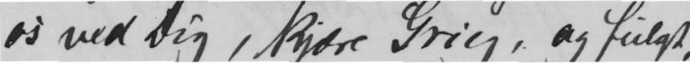os ved Dig, gjære Grieg, og fulgt
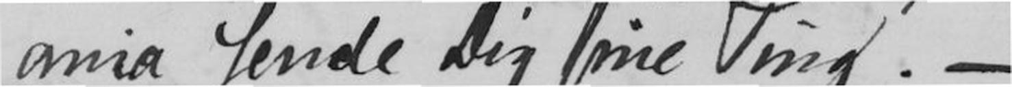ania sende Dig sine Ting. -
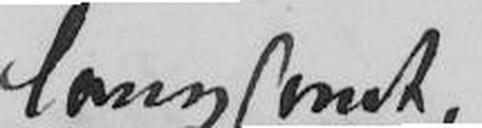langsomt,
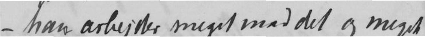- han arbejder meget med det og meget
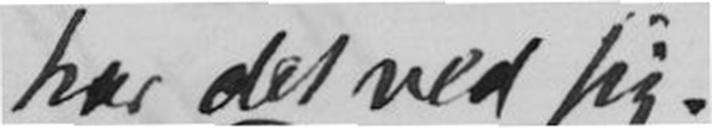har det ved sig.
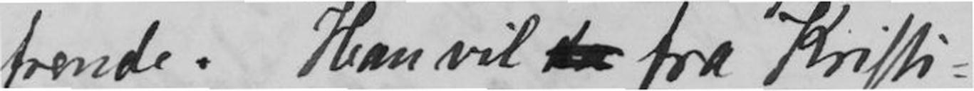trende. Han vil fra Kristi-
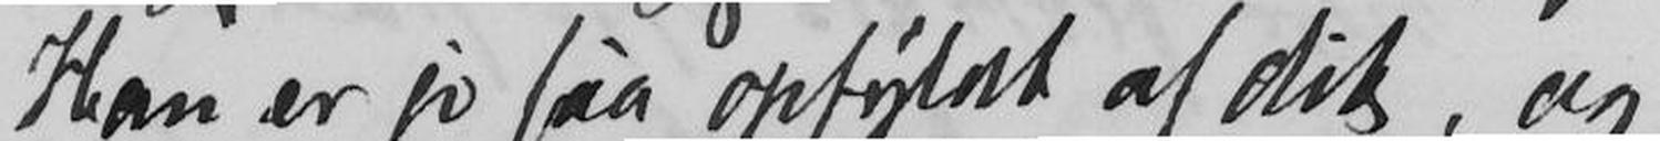Han er jo saa opfyldt af dit, og
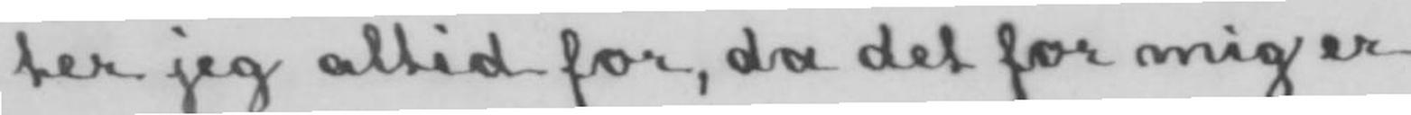ter jeg altid for, da det for mig er
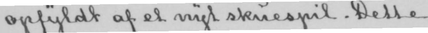opfyldt af et nyt skuespil. Dette
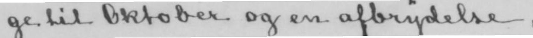ge til Oktober og en afbrydelse,
